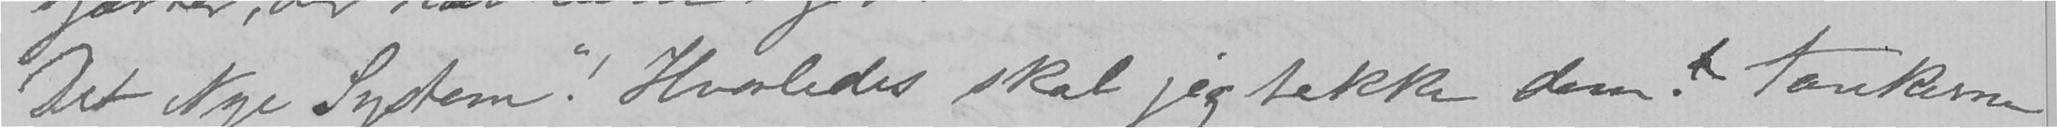"Det Nye System"! Hvorledes skal jeg takke dem! Tankerne
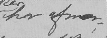har afmer-
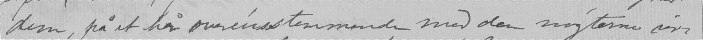dem, på et hår overensstemmende med den nøgterne vir-
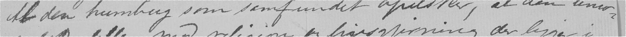Al den humbug som samfundet opelsker, at den univer-
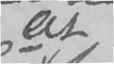at
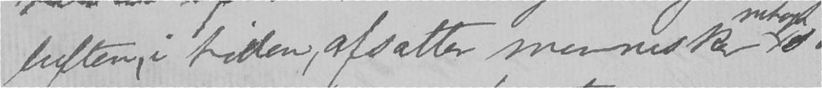luften, i tiden, afsætter menneskene
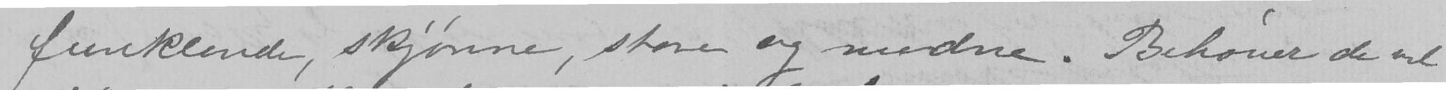funklende, skjønne, store og modne. Behøver de vel
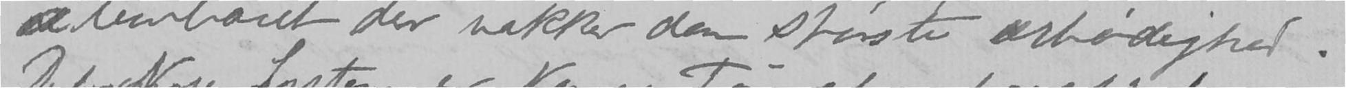åbenbaret der vækker den største ærbødighed.
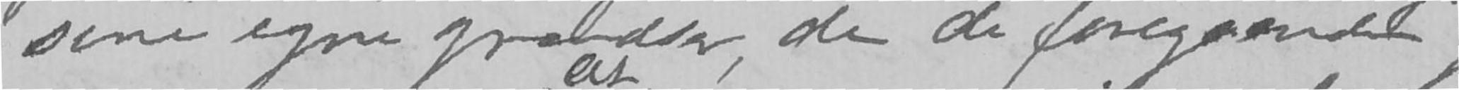sine egne grændse, de de foregående
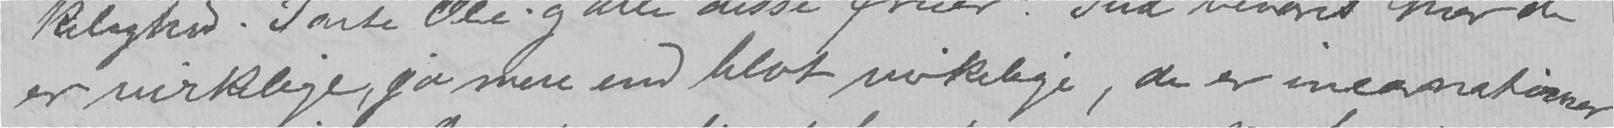er virkelige, ja mere end blot virkelige, de er incarnationer
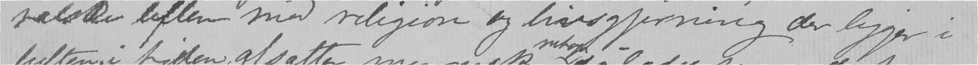salske leften med religion og livsgjerning der ligger i
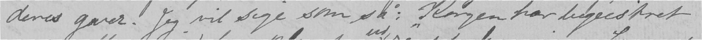deres gaver. Jeg vil sige som så: Kongen har begeistret
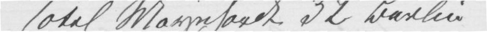Hotel Maynhardt 32 Berlin
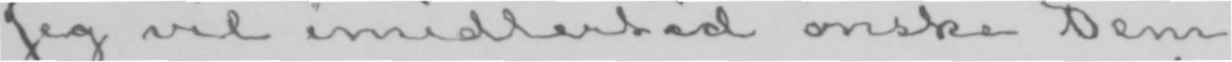Jeg vil imidlertid ønske Dem
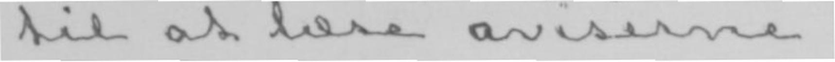til at læse aviserne.
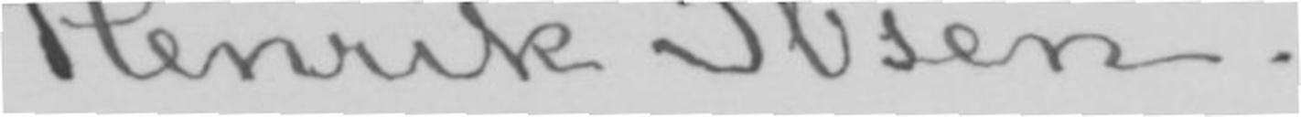Henrik Ibsen.
